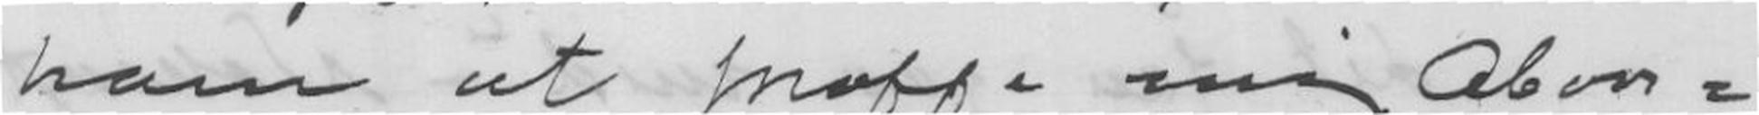ham at skaffe mig Abon-
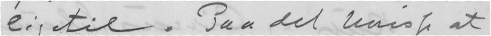ligetil. Paa det uvisse at
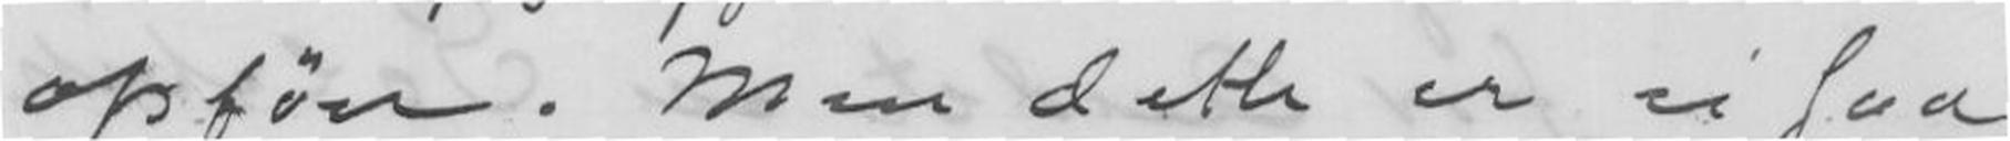opføre. Men dette er ei saa
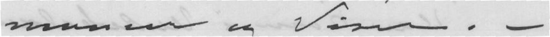manser og viser.-
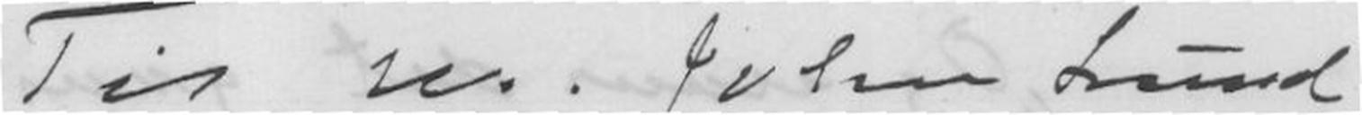Til hr. Johan Lund
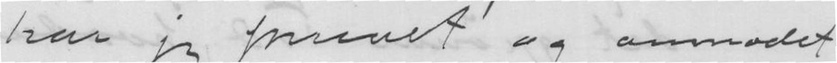har jeg skrevet og anmodet
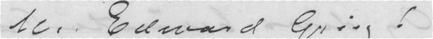Hr. Edward Grieg!
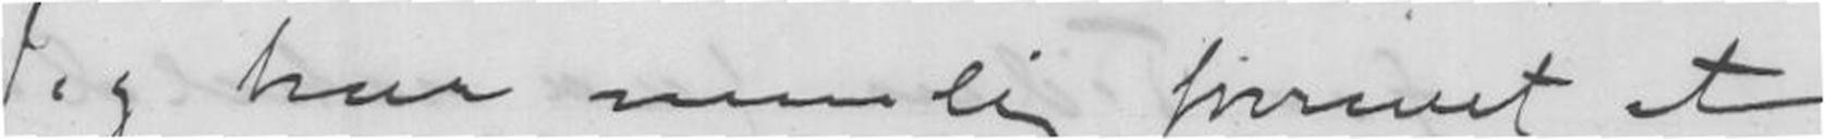Jeg har nemlig skrevet et
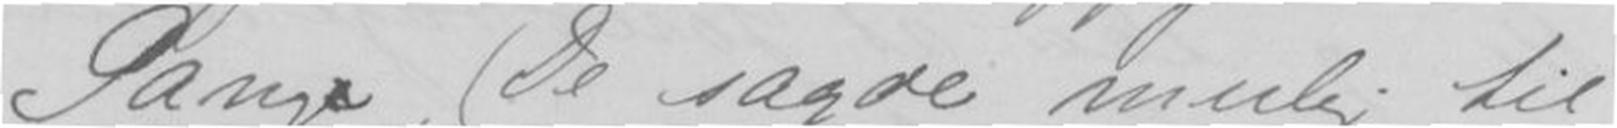Gang, (De sagde mulig til
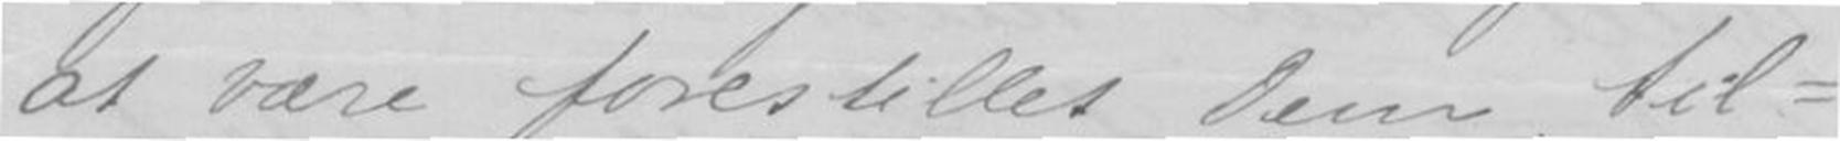at være forestillet Dem, til-
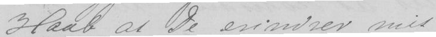Haab at De erindrer mit
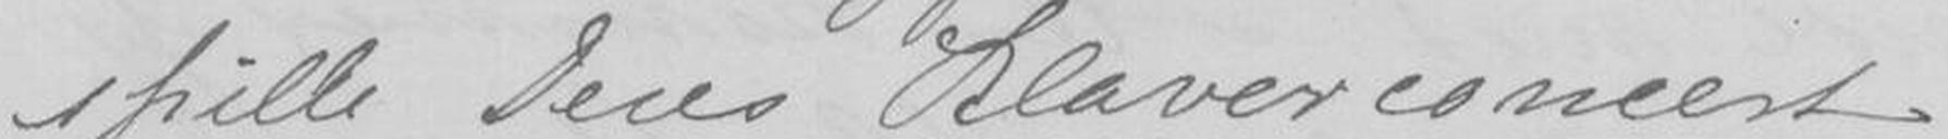spille Deres Klaverconcert
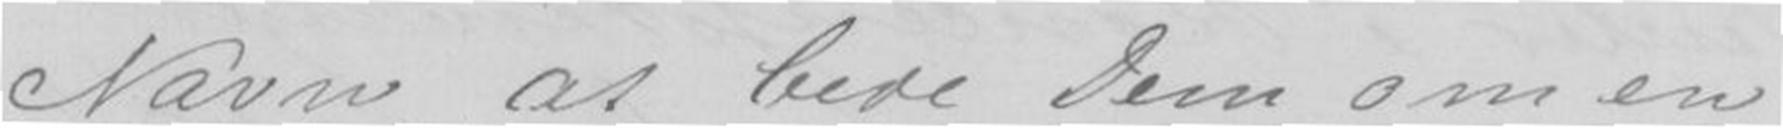Navn, at bede Dem om en
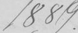1889
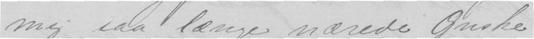mig saa længe nærede Ønske
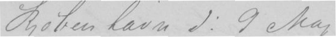København d. 9 Mai
